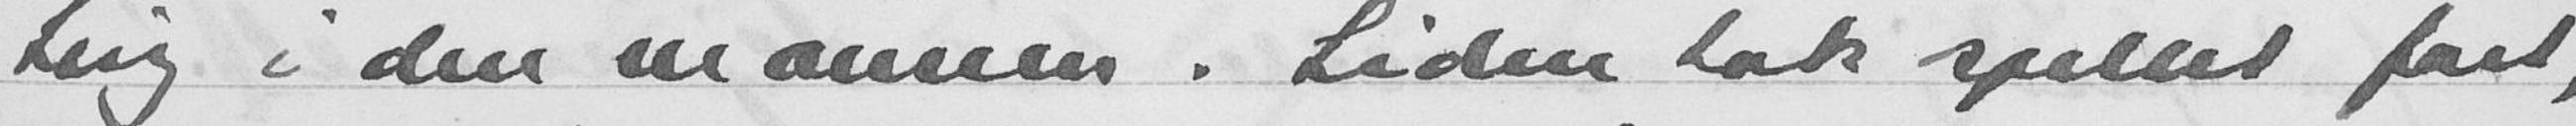ting i den mannen. Siden tok spillet fart,
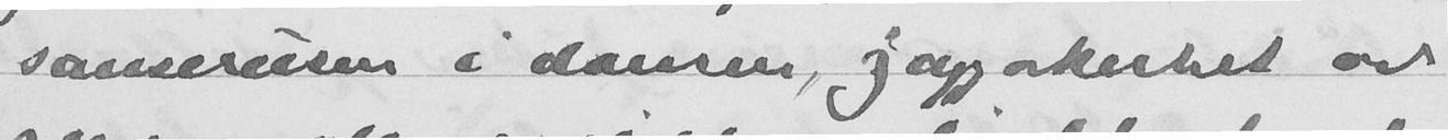sanserusen i dansen, jagorkeshet av
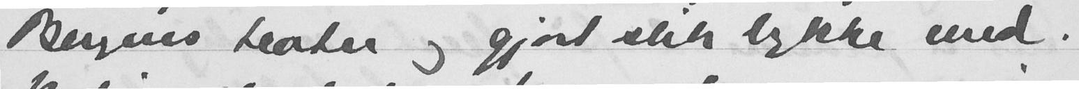Bergens teater og gjort slik lykke med.
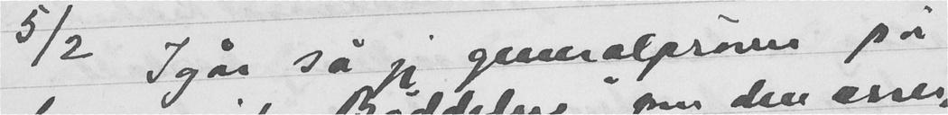5/2 Igår så jeg generalprøver på
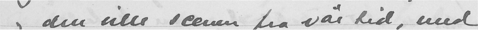og den ville scene fra vår tid, med
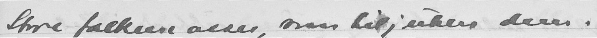Store folkemasser, som tiljubler dem.
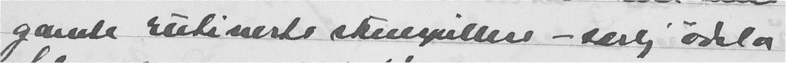gamle Euliverte skuespillere - særlig ødela
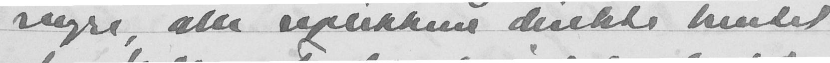seipre, alle replikkene direkte hentet
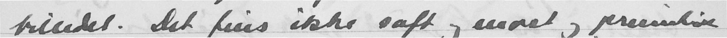billedet. Det fins ikke saft og most og prumitie
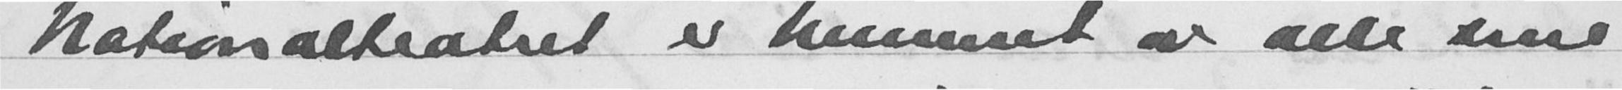Nationalteatret er hemmet av alle sine
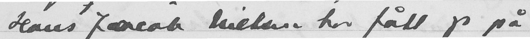Hans Jacob Nilsen har fått op på
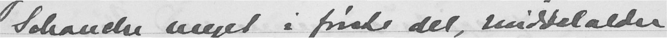Schauche meget i første del, middelalder
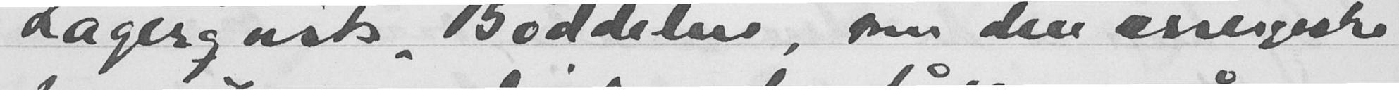Lagerqvists Bøddelen, som den energiske
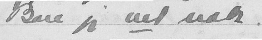Bare jeg vet nok.
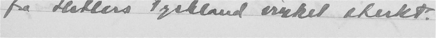fra Hitlers Tyskland virket sterkt
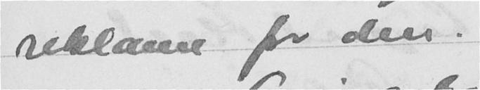reklame for den.
